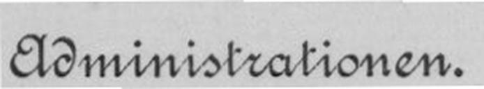Administrationen.
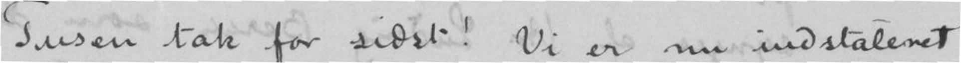Tusen tak for sidst! Vi er nu indstaleret
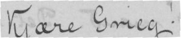Kjære Grieg!
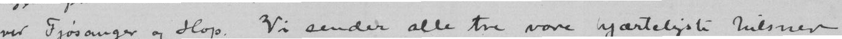ved Fjøsanger og Hop. Vi sender alle tre vore hjærteligste hilsner
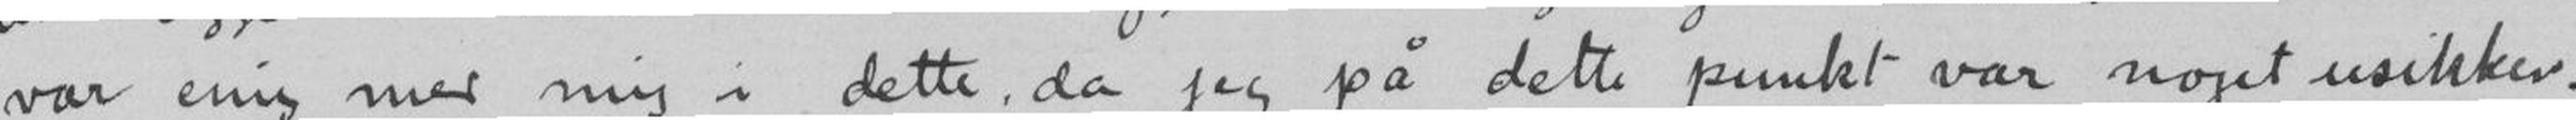var enig med mig i dette, da jeg på dette punkt var noget usikker.
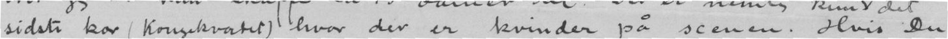sidste kor (Kongekvartet) hvor der er kvinder på scenen. Hvis du
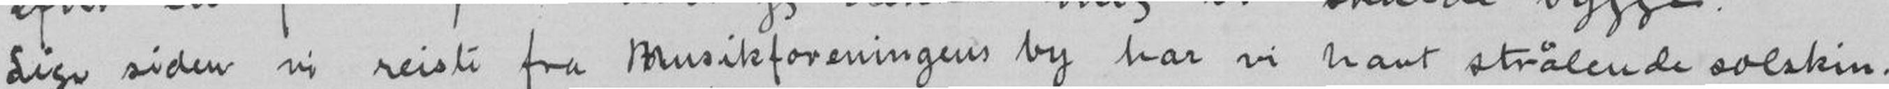Lige siden vi reiste fra Musikforeningens by har vi havt strålende solskin.
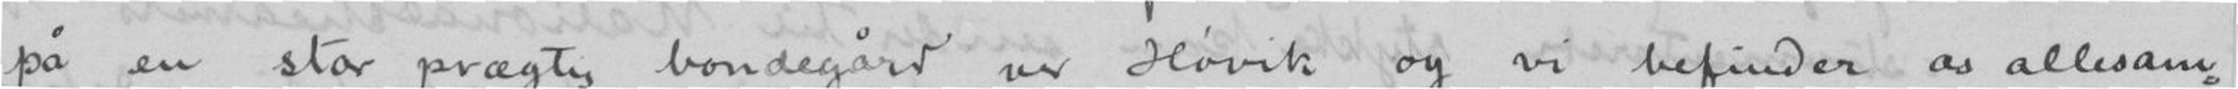på en stor prægtig bondegård ved Høvik og vi befinder os allesam-
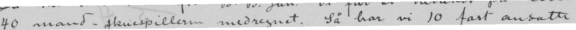40 mand - skuespillerne medregnet. Så har vi 10 fast ansatte
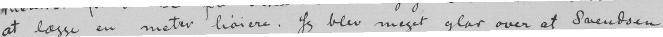at lægge en meter høiere. Jeg blev meget glad over at Svendsen
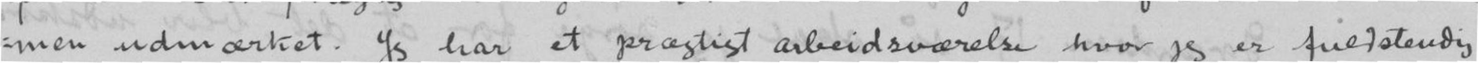men udmærket. Jeg har et prægtigt arbeidsværelse hvor jeg er fuldstendig
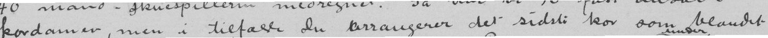kordamer, men i tilfælde du arrangerer det sidste kor som blandet
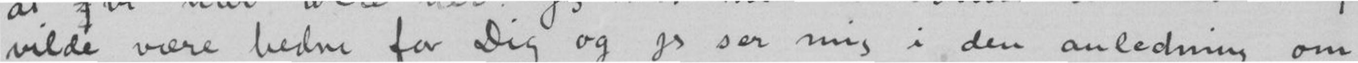vilde være bedre for Dig og jeg ser mig i den anledning om
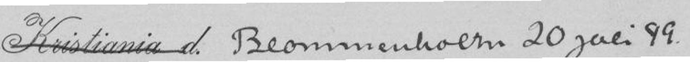Kristiania d. Blommenholm 20 juli 99
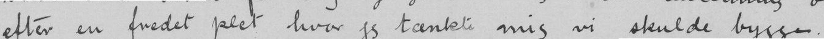efter en fredet plet hvor jeg tænkte mig vi skulde bygge.
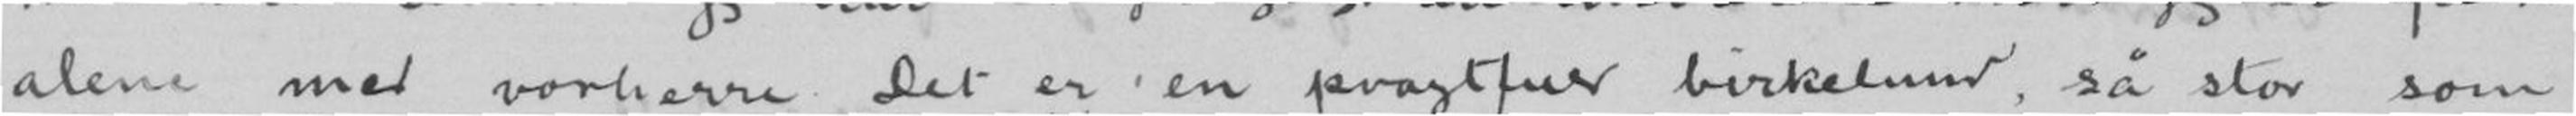alene med vorherre. Det er en pragtfuld birkelund, så stor som
